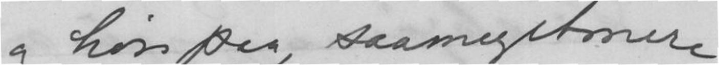og høre paa, saa meget mere
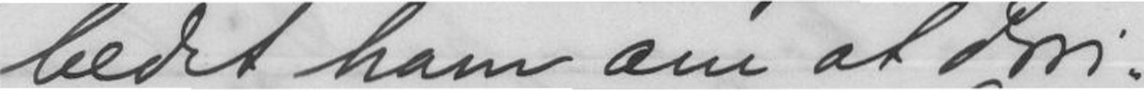bedet ham om at diri-
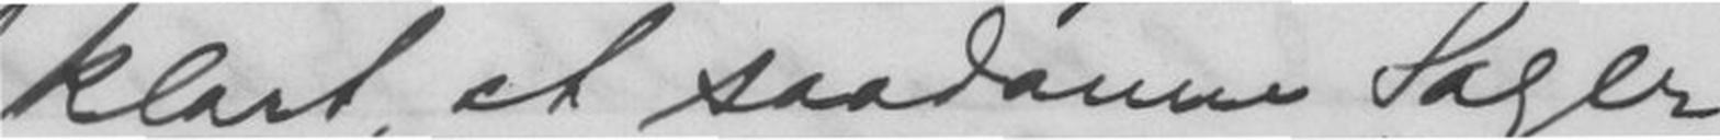klart, at saadanne Sager
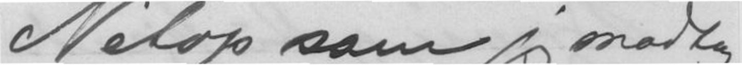Netop som jeg modtog
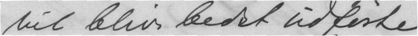vil blive bedst udførte
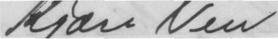Kjære Ven!
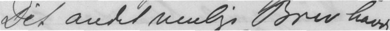Dit andet venlige Brev havde
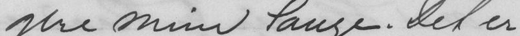gere min Sange. Det er
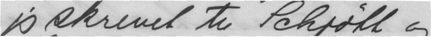jeg skrevet til Schjøtt og
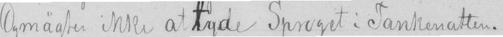Og mägter ikke at tyde Sproget i Tankenatten.
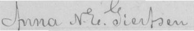Anna N. E. Giertsen
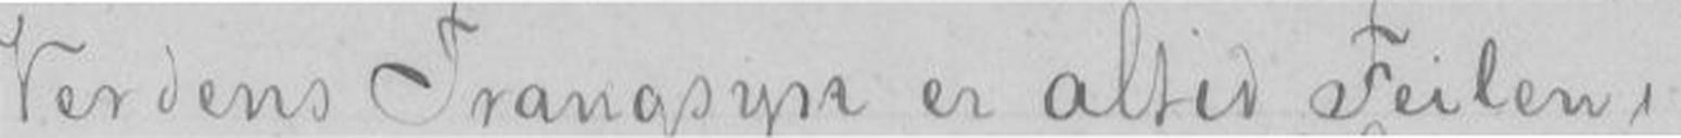Verdens Trangsyn er altid Feilen.
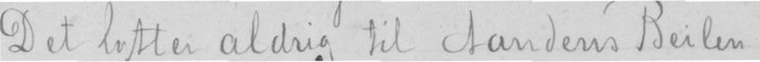Det lytter aldrig til Aandens Beilen
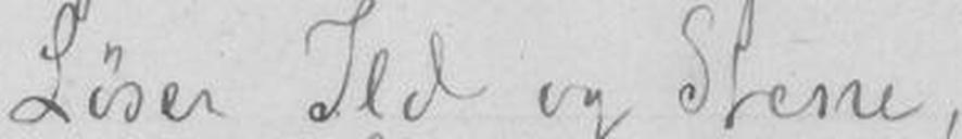Løser Ild og Stene,
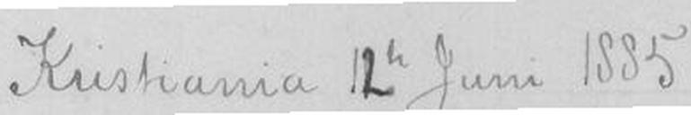Kristiania 12te Juni 1885
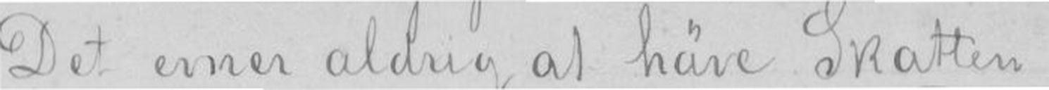Det evner aldrig at häve Skatten
